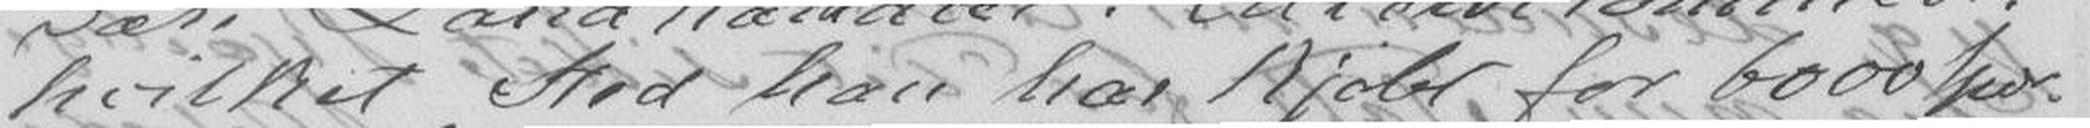hvilket Sted han har kjøbt for 6000 Spd..
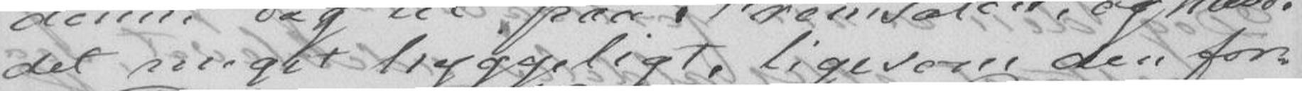det meget hyggeligt, ligesom den for-
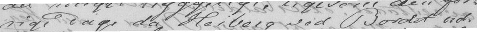rige Dage da Heiberg ved Bordet ud-
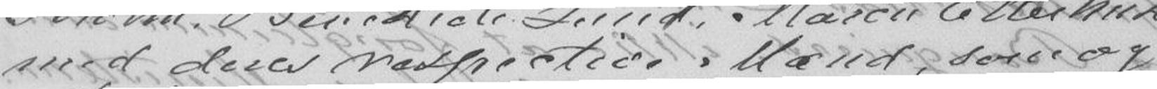med deres respective Mand, som og
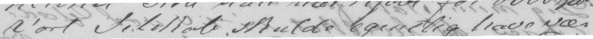Vort Selskab skulde egentlig have vær-
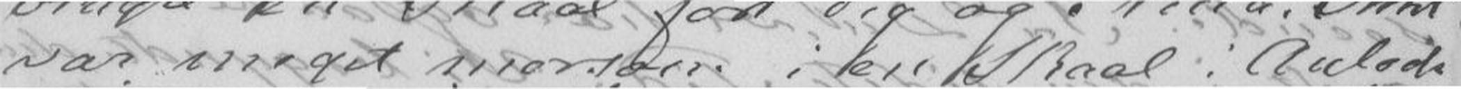var meget morsom i en Skaal i Anled-
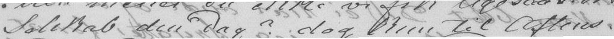selskab den Dag? dog kun til Aftens.
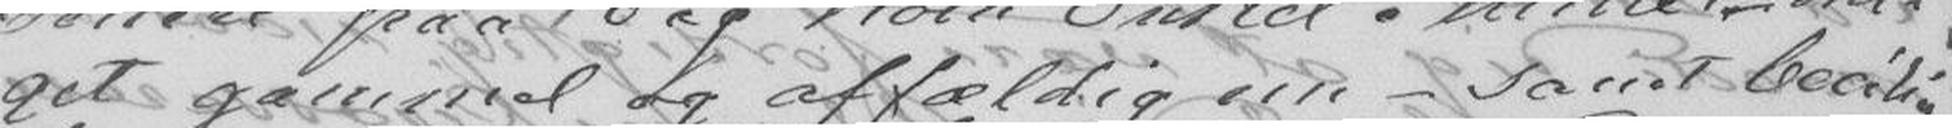get gammel og affældig nu - samt Cecilie
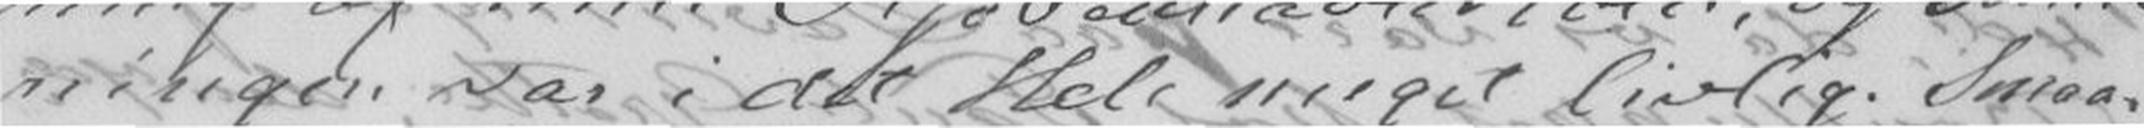ningen var i det Hele meget livlig. Smaa-
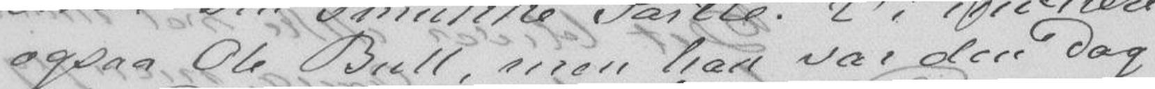ogsaa Ole Bull, men han var den Dag
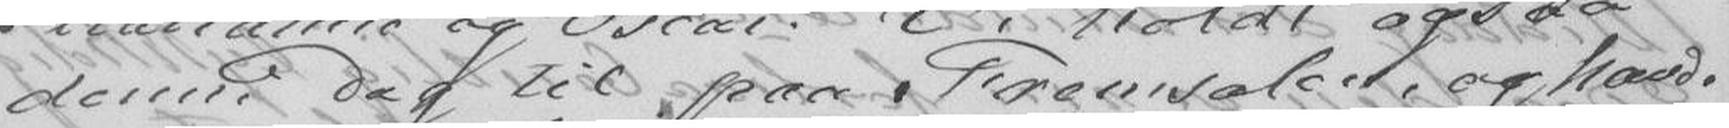denne Dag til paa Fremsalen, og havde
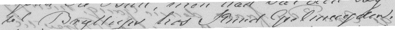til Bryllups hos Knud Geelmuyden.
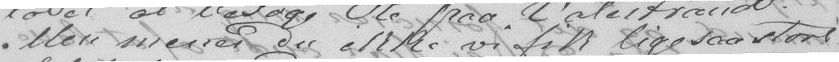Men mener Du ikke vi fik ligesaa stort
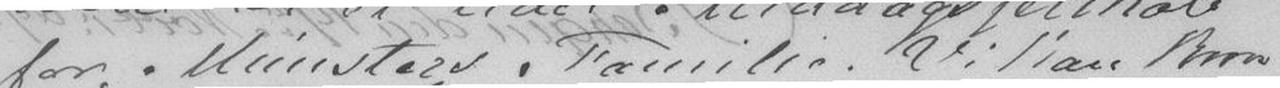for Münsters Familie. Vi kan kun
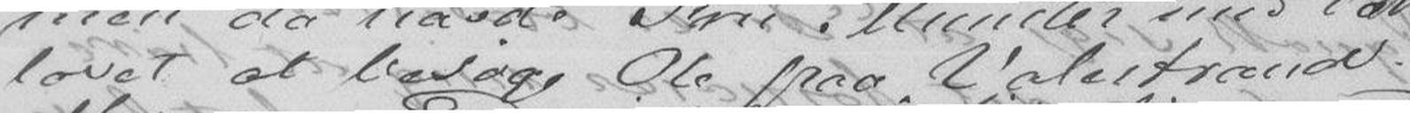lovet at besøge Ole paa Valestrand.
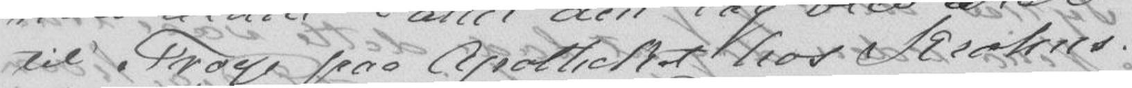til Troye paa Apotheket hos Krohns.
